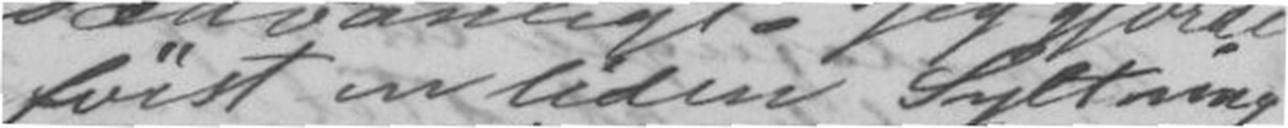først en liden Syltning
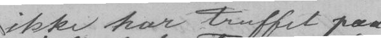ikke har truffet paa
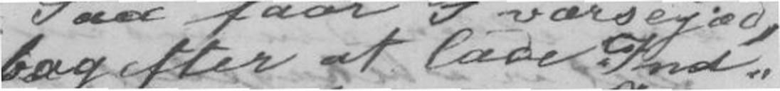bagefter at lade Ind-
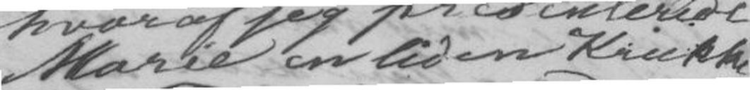Marie en liden Krukke
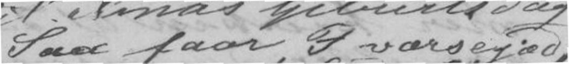Saa faar I værsegod,
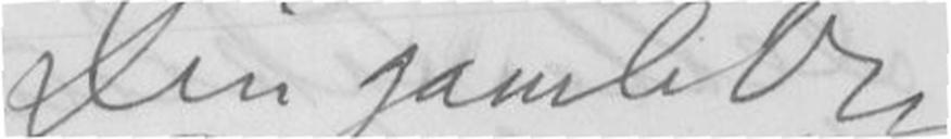Din gamle Ven
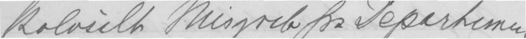kolosalt Misgreb fra Departemen-
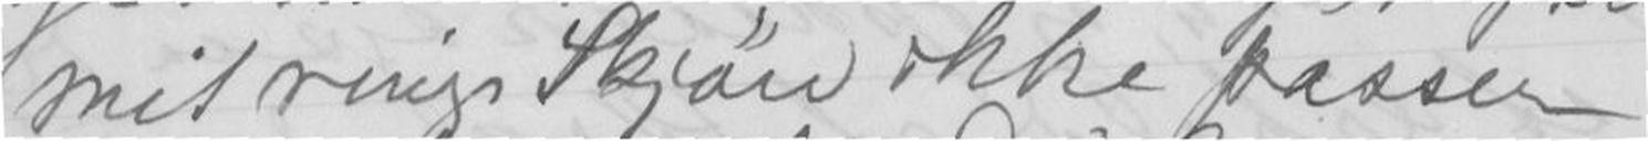mit ringe Skjøn ikke passer.
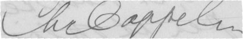Chr Cappelen
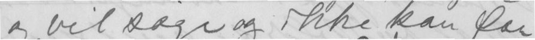og vil søge og ikke kan faa
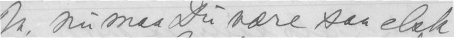Ja, nu maa Du være saa elsk-
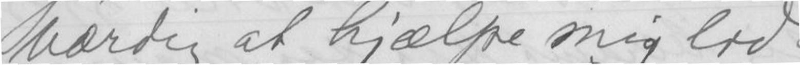værdig at hjælpe mig lidt
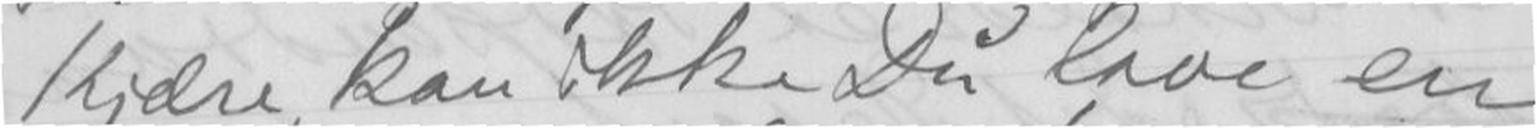Kjære, kan ikke Du lave en
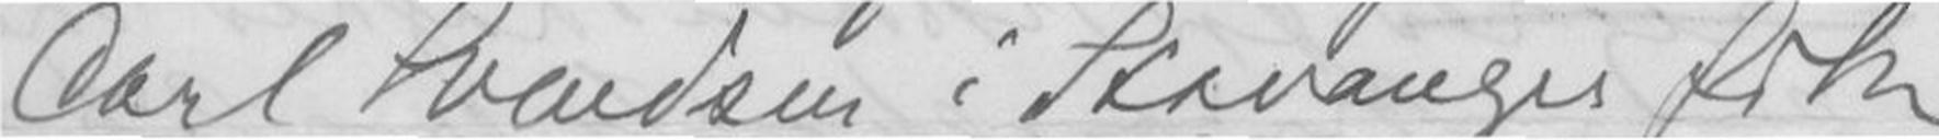Carl Svendsen i Stavanger fik
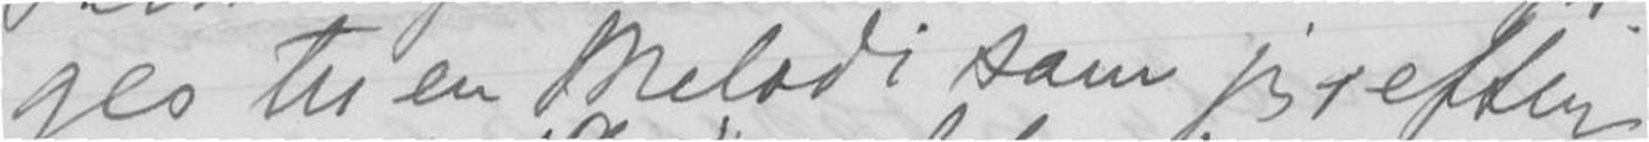ges til en Melodi som jeg, efter
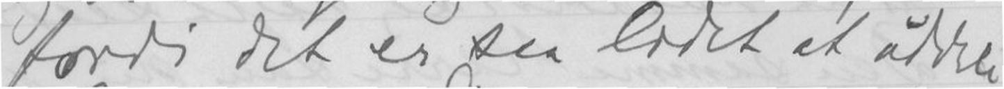fordi det er saa lidet at uddele.
